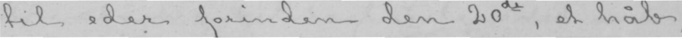til eder forinden den 20de, et håb,
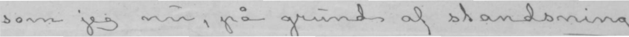som jeg nu, på grund af standsning
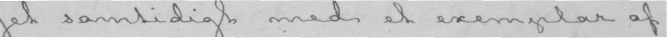get samtidigt med et exemplar af
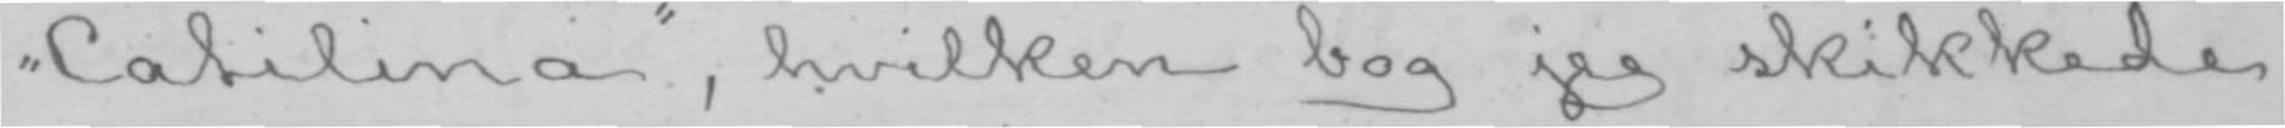«Catilina», hvilken bog jeg skikkede
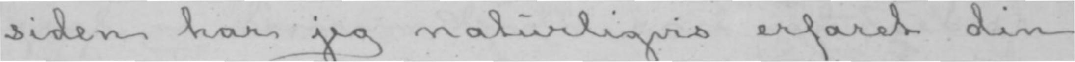siden har jeg naturligvis erfaret din
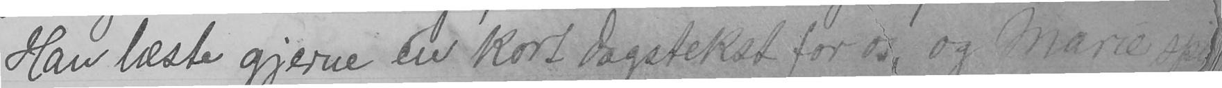Han læste gjerne en kort dagstekst for os, og Marie spil-
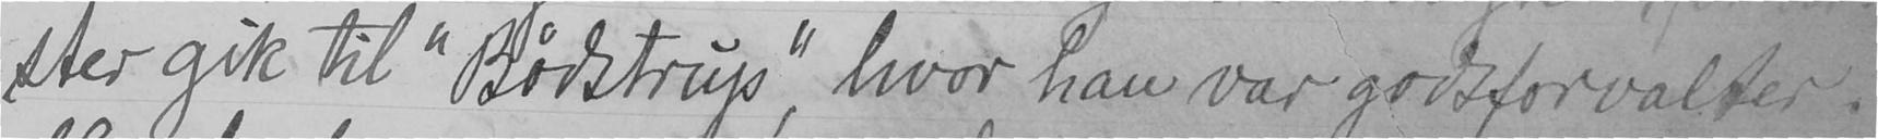ster gik til "Bødstrup", hvor han var godsforvalter.
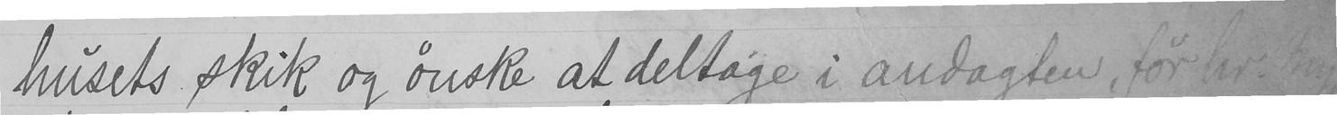husets skrik og ønske at deltage i andagten, før hr. Myn-
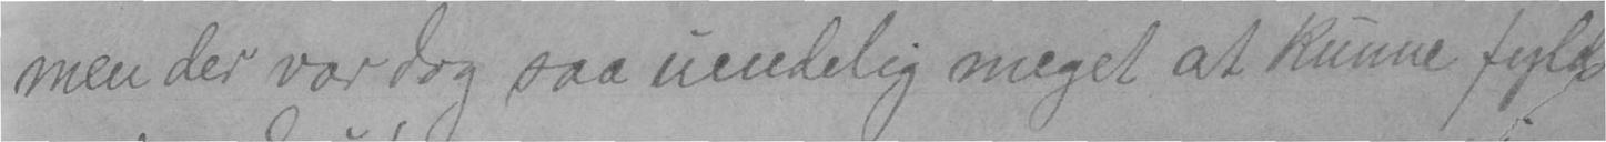men der var dog paa uendelig meget at kunne fyld
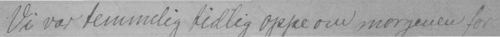Vi var temmelig tidlig oppe om morgenen for
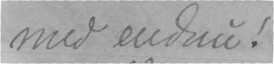med endnu!
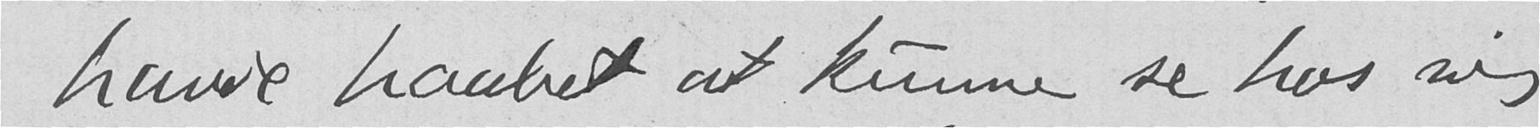havde haabet at kunne se hos sig
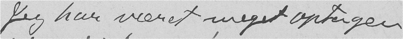Jeg har været meget optagen
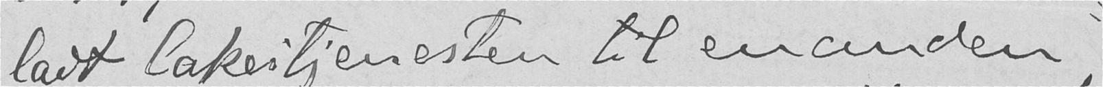ladt lakeitjenesten til en anden,
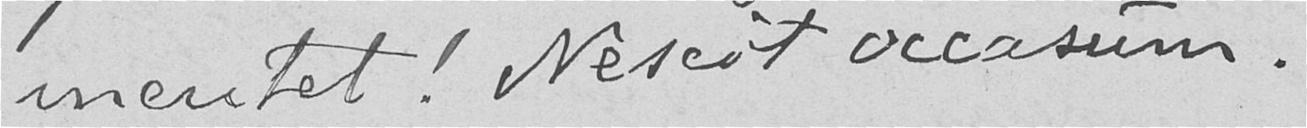mentet! Nescit occasum.
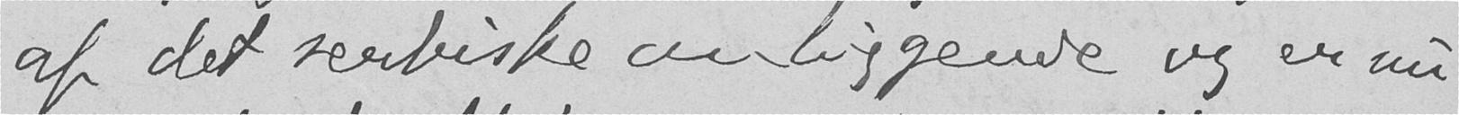af det serbiske anliggende og er nu
